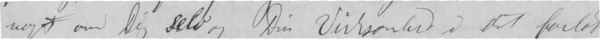noget om Dig selv og Din Virksomhed i det forløb-
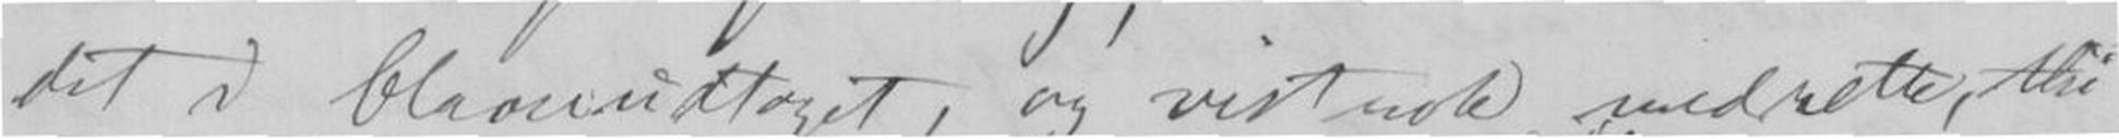det i Claverudtaget, og vistnok medrette, thi
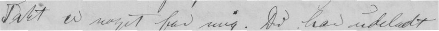Takt er noget for mig; Du har udeladt
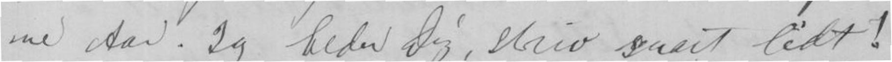ne Aar. Jeg beder Dig, skriv snart lidt!
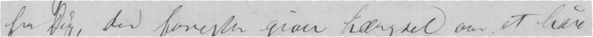fra Dig, den foresten giver Længsel om at høre
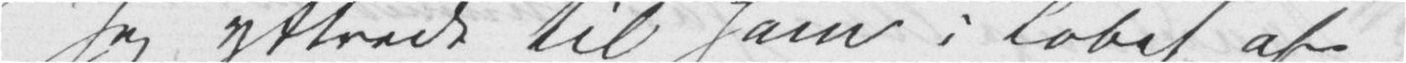Jeg yttrede til ham i Løbet af
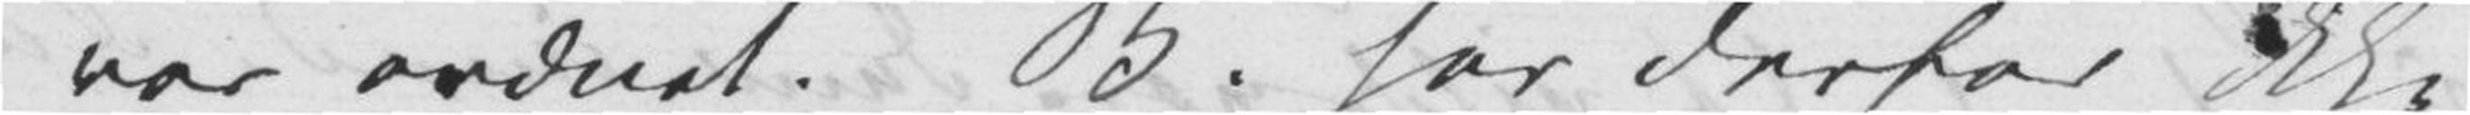var ordnet. B. har derfor ikke
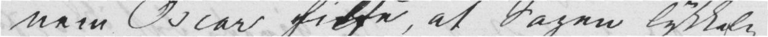nem Oscar hilse, at Sagen lykkelig
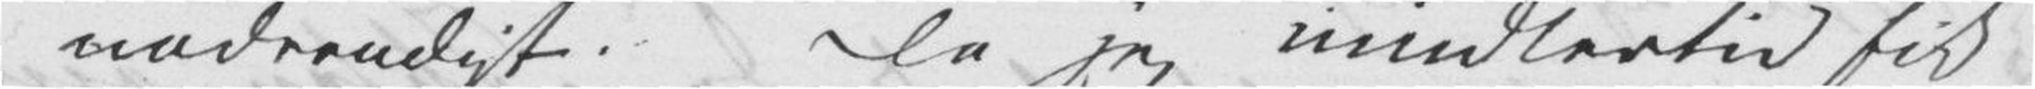nødvendigt. Da jeg imidlertid fik
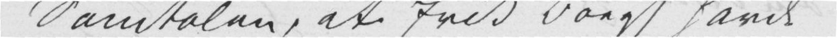Samtalen, at Erik Bøegh havde
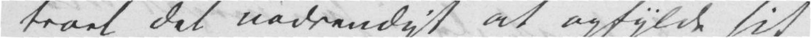troet det nødvendigt at opfylde sit
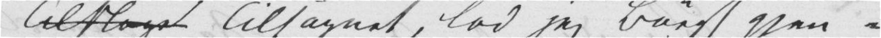Tilslaget Tilsagnet, lod jeg Bøegh gjen¬
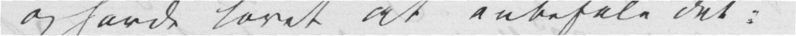og havde lovet at anbefale det.
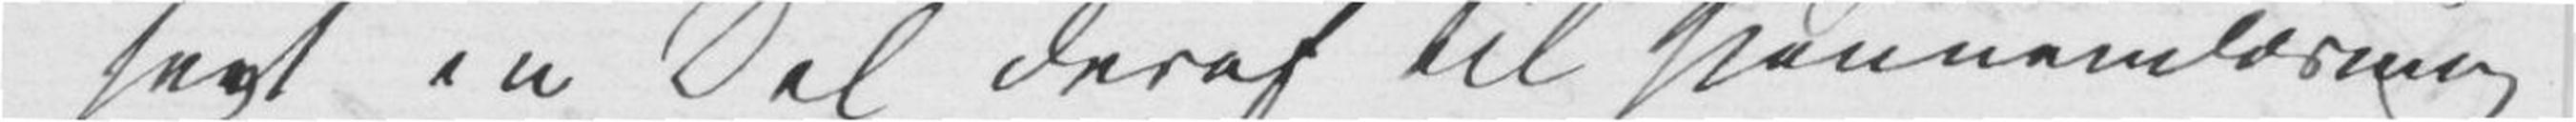havt en Del deraf til Giennemlæsning
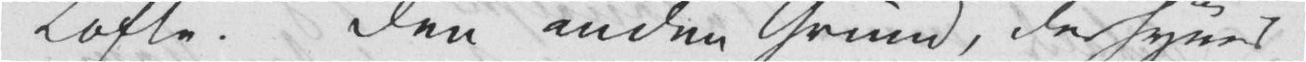Løfte. Den anden Grund, der synes
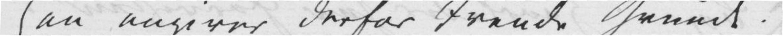Han angiver derfor tvende Grunde.
